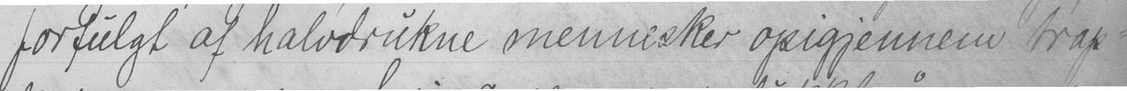forfulgt af halvdrukne mennesker opigjennem trap-
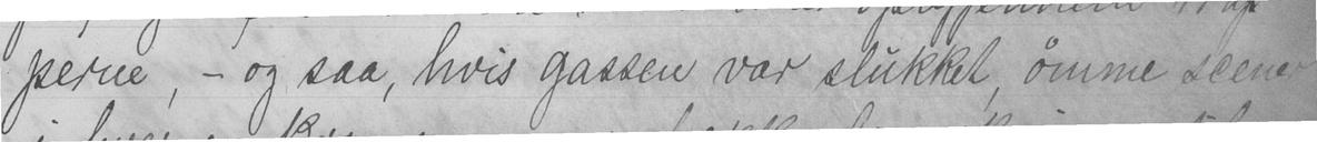perne, - og saa, hvis gassen var slukket, ømme scener
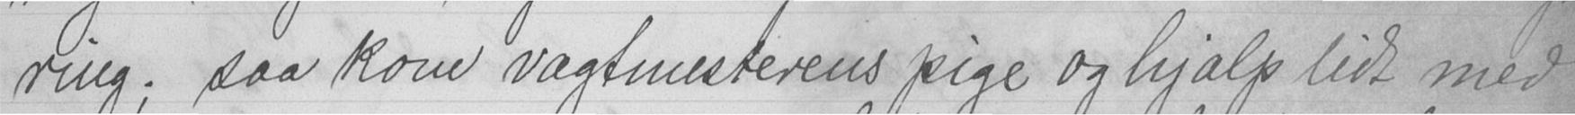ring; saa kom vagtmesterens pige og hjalp lidt med
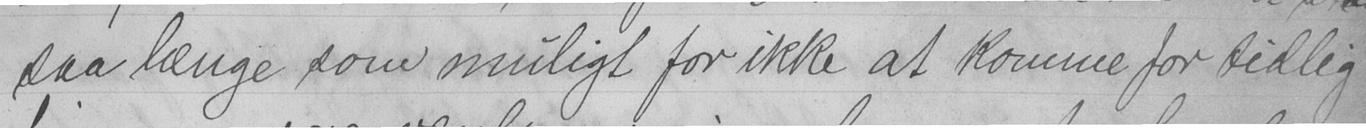saa lenge som muligt for ikke at komme for tidlig
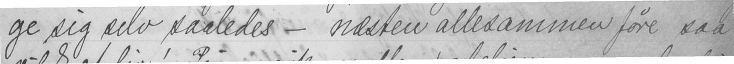ge sig selv saaledes - næsten allesammen føre saa
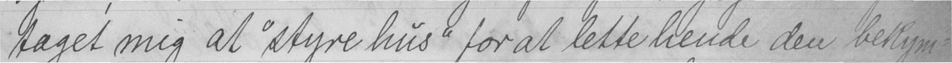taget mig at "styre hus" for at lette hende den bekym-
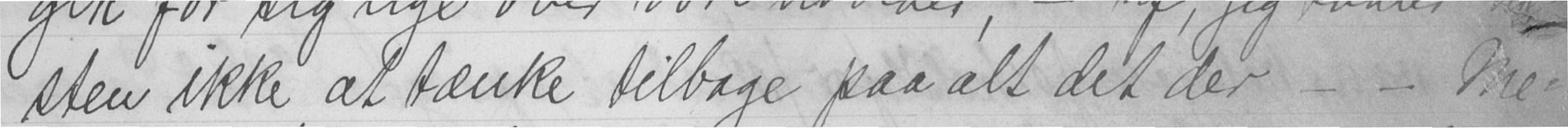sten ikke at tænke tilbage paa alt det der - - Me-
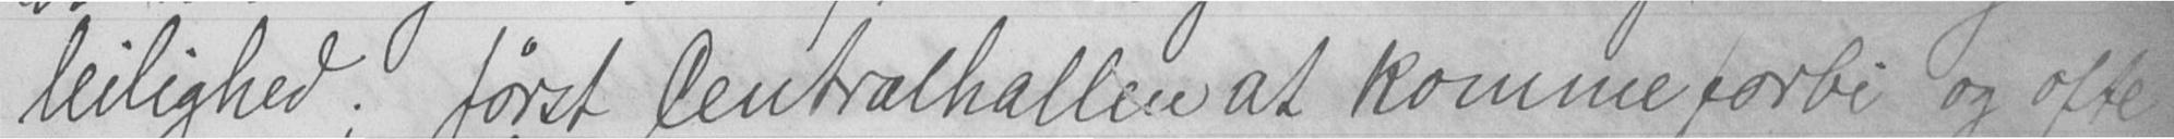leilighed; først Centralhallen at komme forbi og ofte
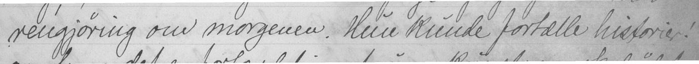rengjøring om morgenen. Hun kunde fortælle historier!
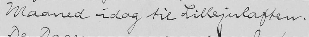Maaned idag til Lillejulaften.
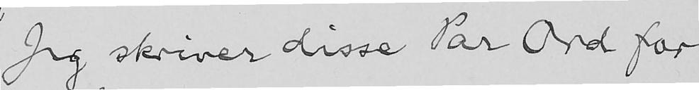Jeg skriver disse Par Ord for
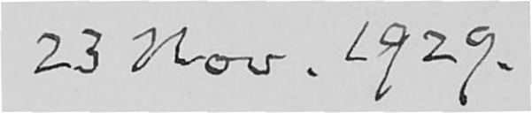23 Nov. 1929.
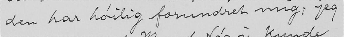den har høilig forundret mig, jeg
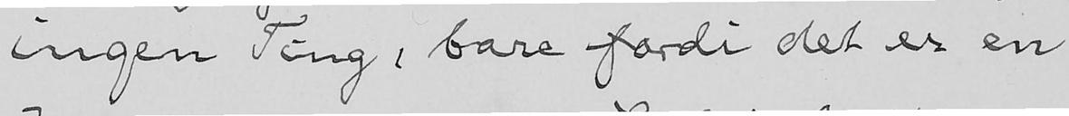ingen Ting, bare fordi det er en
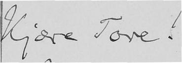Kjære Tore!
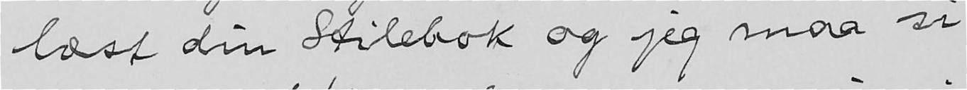læst din Stilebok og jeg maa si
